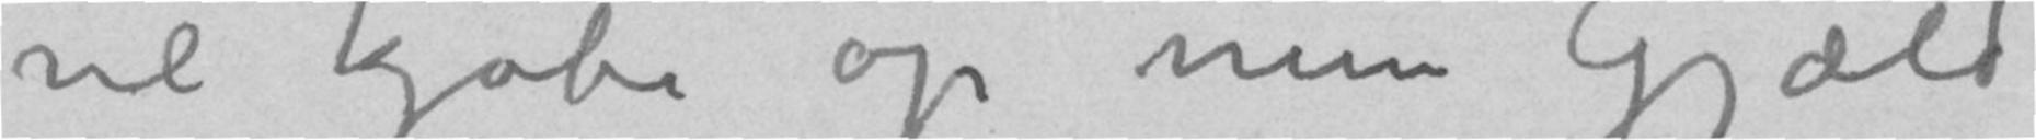vil kjøbe op min Gjæld
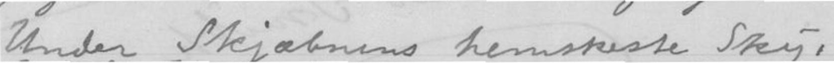Under Skjæbnes hemskeste Sky,
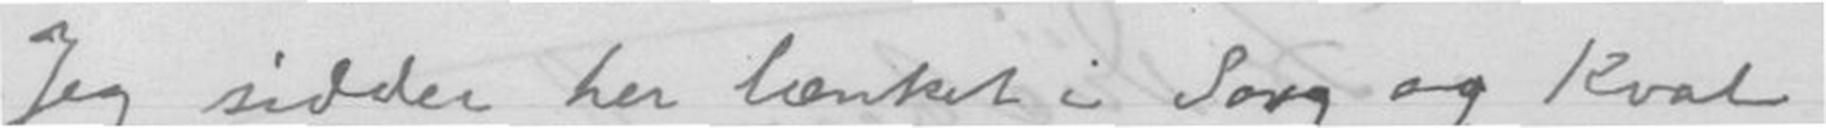Jeg sidder her lænket i Sorg og Kval
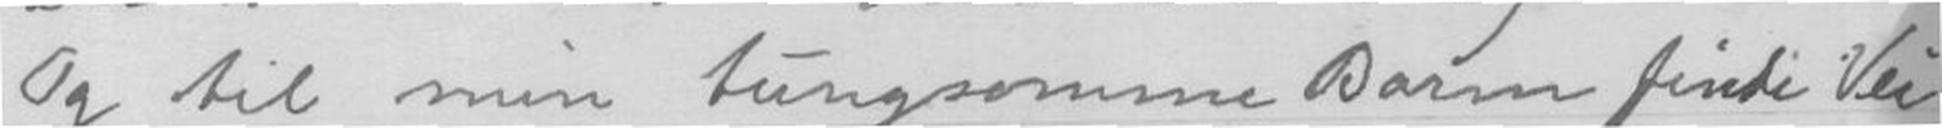Og til min tungsomme Barm findi Vei
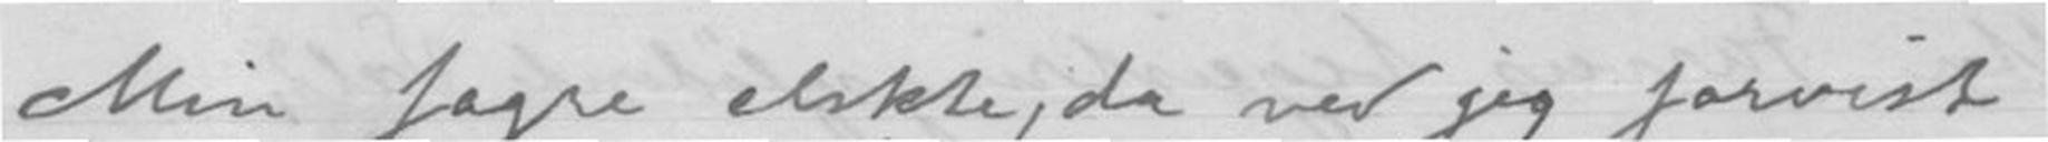Min fagre elskte, da ved jeg forvist
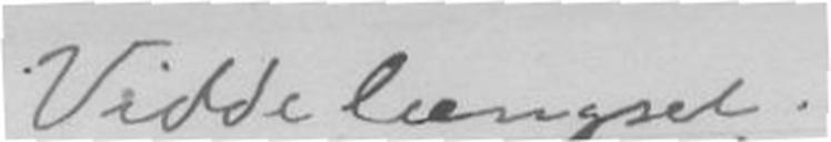Viddelængsel.
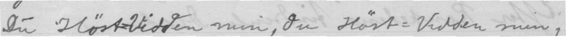Du Høst-Vidden min, Du Høst-Vidden min,
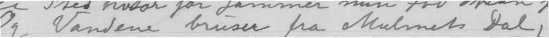Og Vandene bruser fra Mulmets Dal,
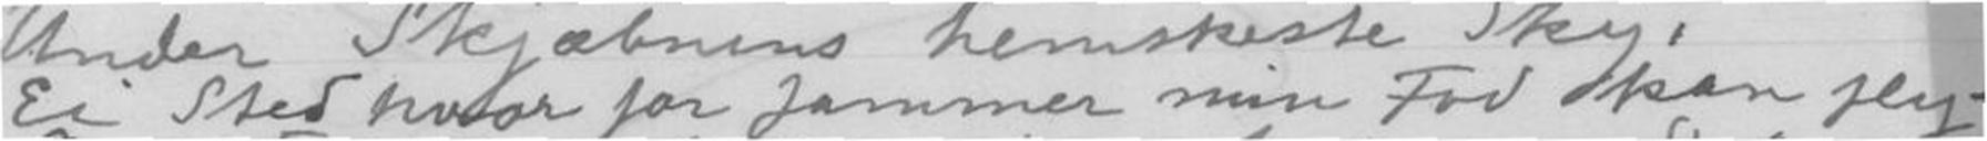- Ei Sted hvor for Jammer min Fod kan fly-
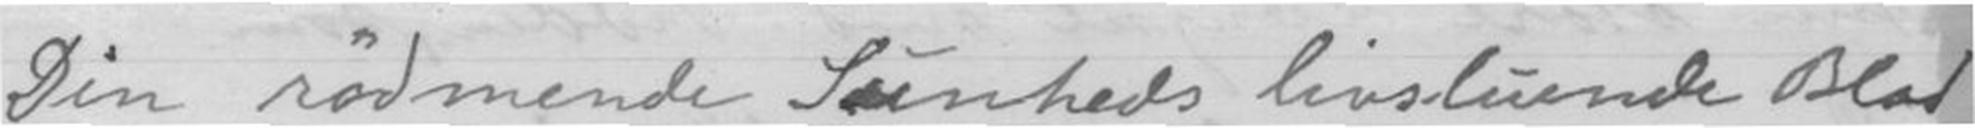Din rødmende Sunheds livsluende Blad
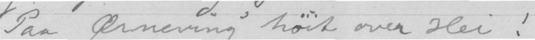Paa Ørneving høit over Hei!
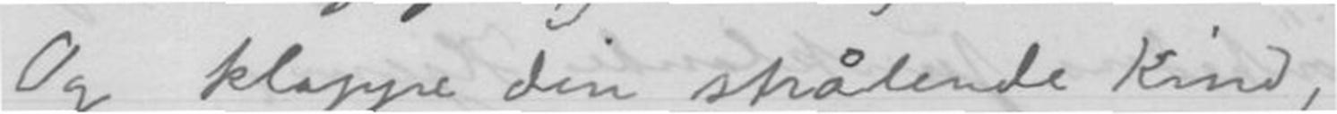Og klappe din strålende kind,
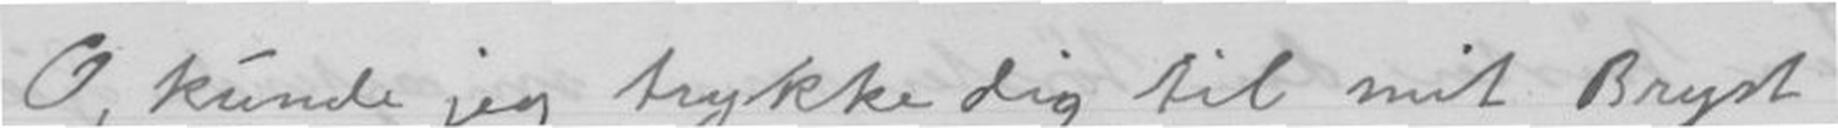O, kunde jeg trykke dig til mit Bryst
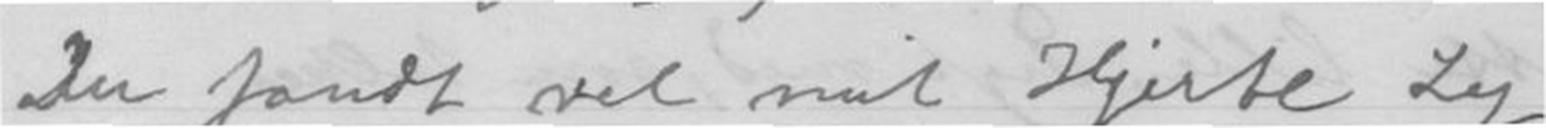Du fandt vel mit Hjerte Ly!
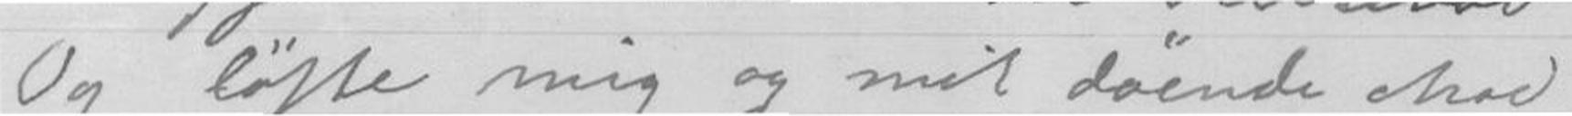Og løfte mig og mit døende Mod
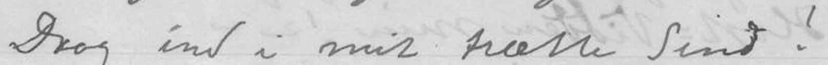Drog ind i mit trætte Sind!
